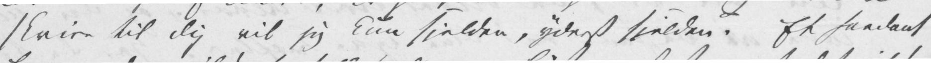skrive til Dig vil jeg kun sjelden, yderst sjelden. Et saadant
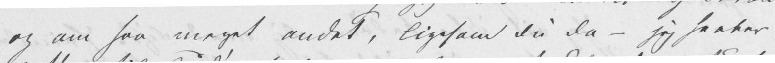og om saa meget andet, ligesom Du da – jeg haaber
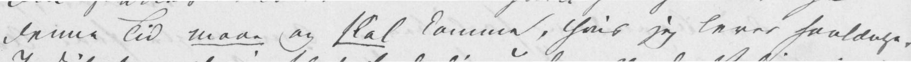denne Tid maae og skal komme, hvis jeg lever saalænge.
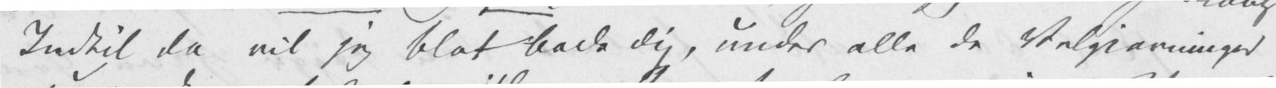Indtil da vil jeg blot bede Dig, under alle de Velgjerninger
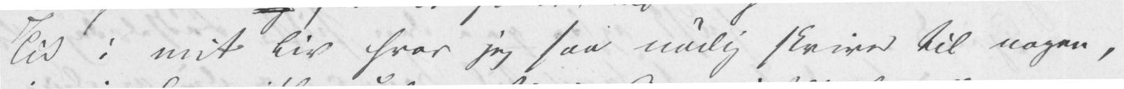Tid i mit Liv hvor jeg saa nødig skriver til nogen,
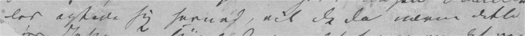der agtede sig herned, vil De da nævne dette
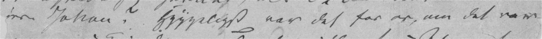ere Johan? Hyggeligst var det for os, om det var
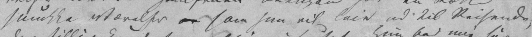smukke Værelser ov som hun vil leie ud til Reisende,
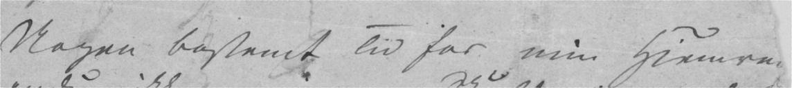Nogen bestemt Tid for min Hjemre
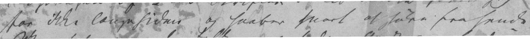for ikke længe siden og haaber snart at høre fra hende
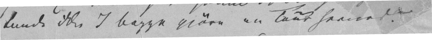Kunde ikke I begge gjøre en Tour herned?
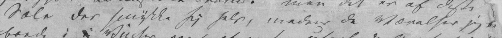Sale der smykke sig selv, medens de Værelser jeg be
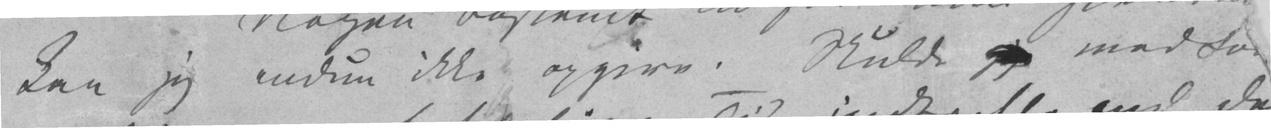kan jeg endnu ikke opgive. Skulde jeg mod Fo
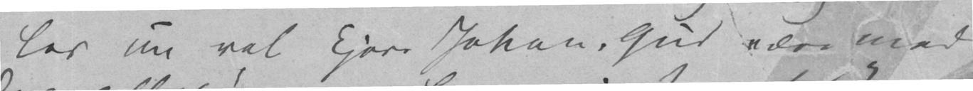Lev nu vel kjere Johan. Gud være med
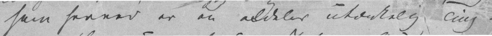ham herned er en aldeles utænkelig Ting.
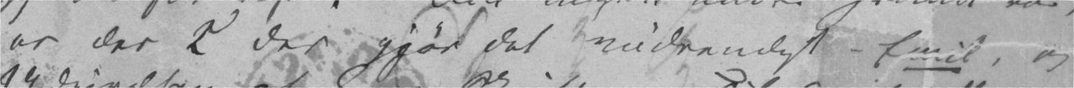er der 2de der gjør det nødvendigt – Emil, og
